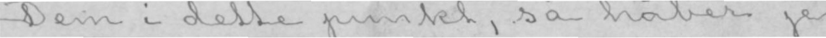Dem i dette punkt, så håber jeg
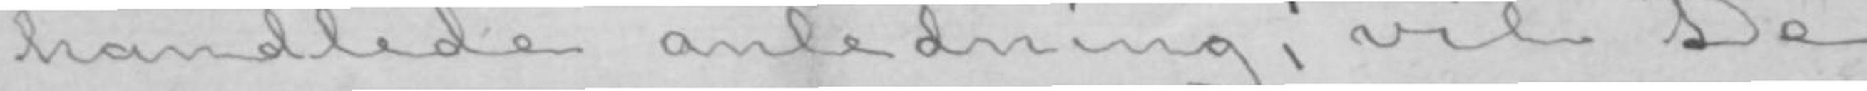handlede anledning, vil De
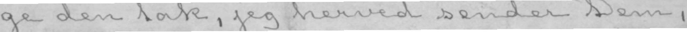ge den tak, jeg herved sender Dem,
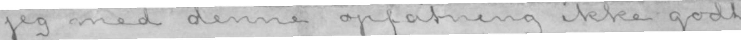jeg med denne opfatning ikke godt
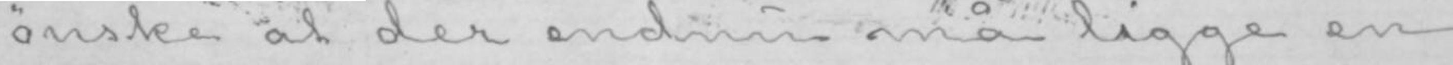ønske at der endnu må ligge en
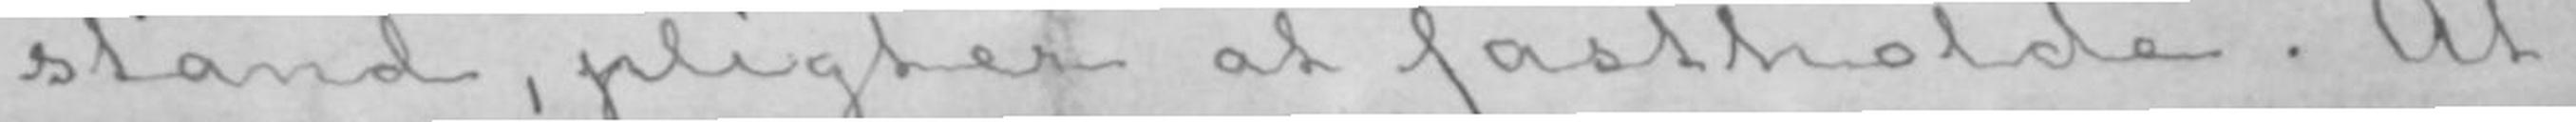stand, pligter at fastholde. At
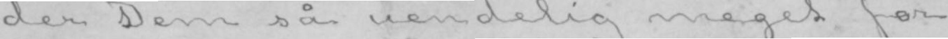der Dem så uendelig meget for
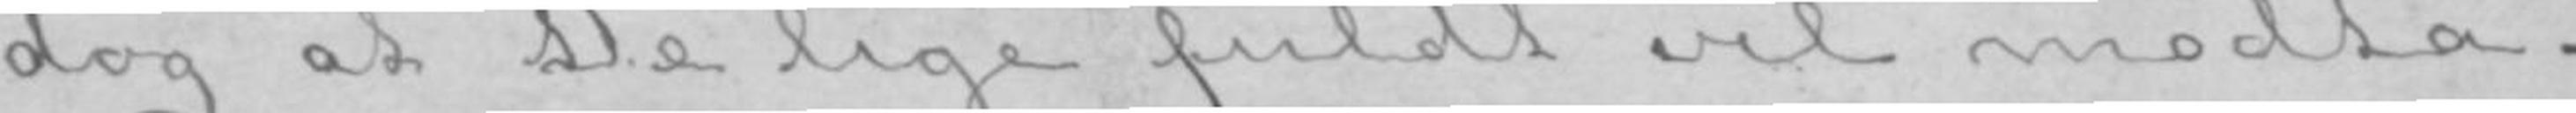dog at De lige fuldt vil modta-
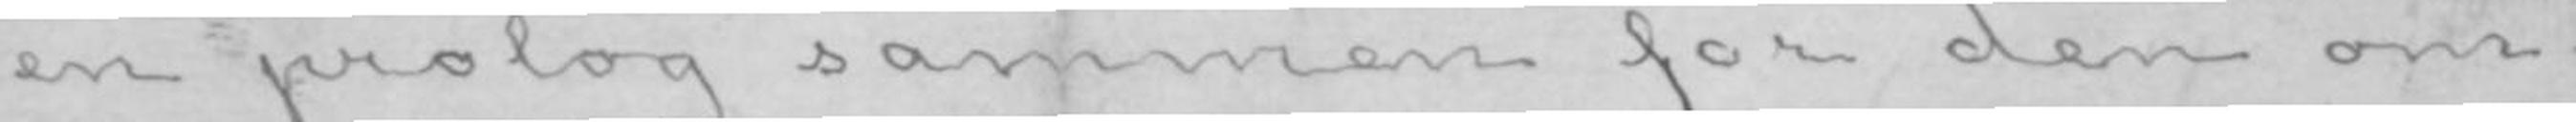en prolog sammen for den om-
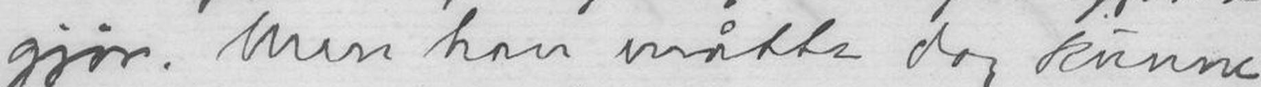gjør. Men han måtte dog kunne
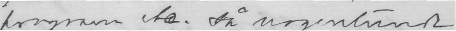program etc. så nogenlunde
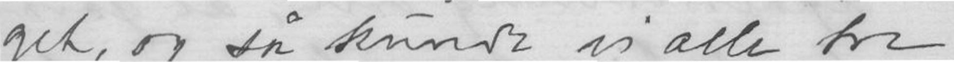get, og så kunde vi alle tre
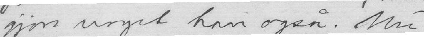gjøre noget han også. Nu
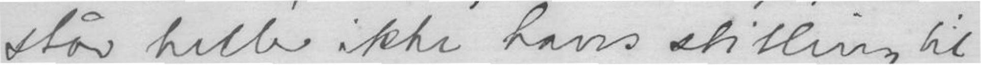står heller ikke hans stilling til
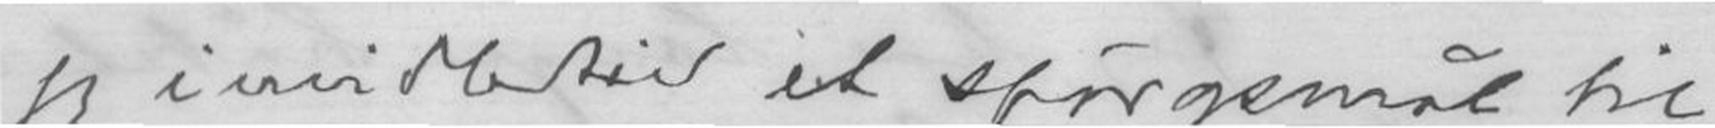jeg imidlertid et spørgsmål til
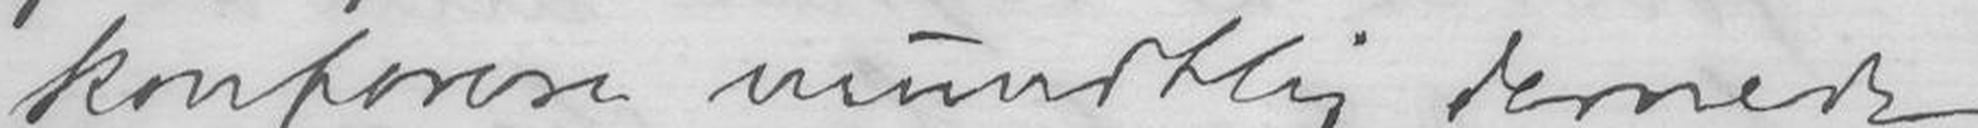konferere muntlig dernede
